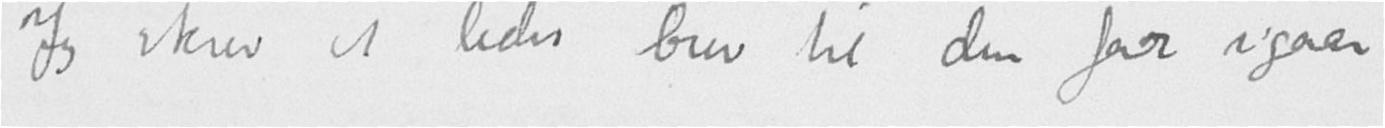Jeg skrev et lidet brev til din far i gaar
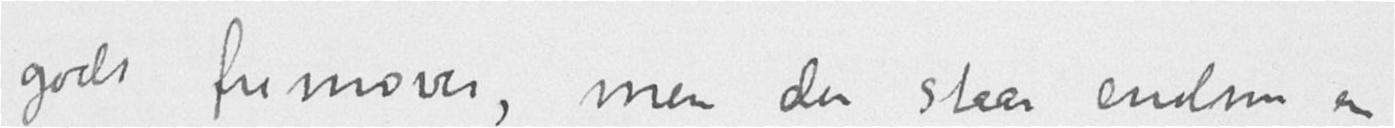godt fremover, men der staar endnu en
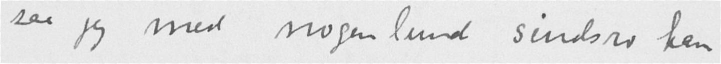saa jeg med nogenlunde sindsro kan
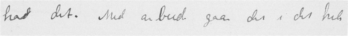had det. Med arbeide gaar det i det hele
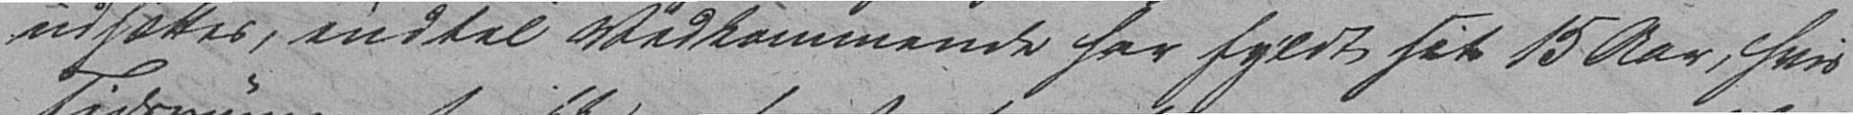udsættes, indtil Vedkommende har fyldt sit 15 Aar, hvis
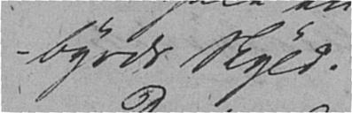byrds Skyld.
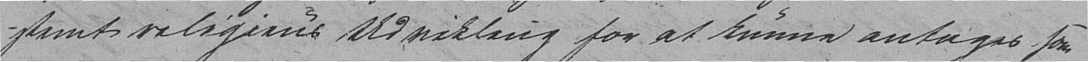stemt religieus Udvikling for at kunne antages som
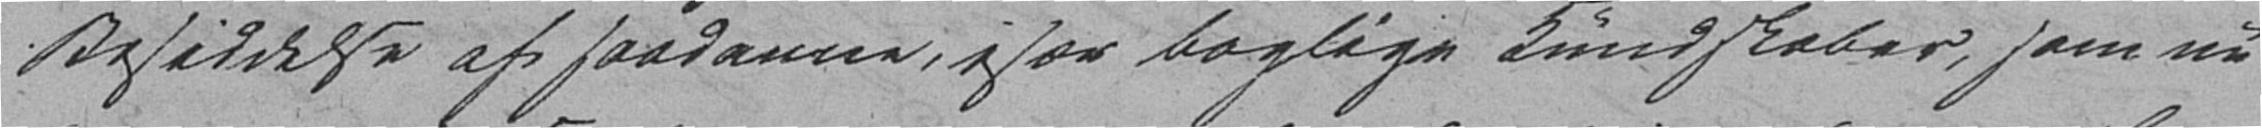Besiddelse af saadanne, især boglige Kundskaber, som nu
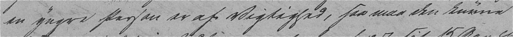en yngre Person er af Vigtighed, saa maa den kunne
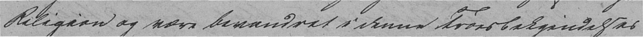Religion og være bevandret i denne Troesbekjendelses
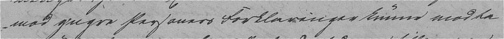mod yngre Personers Forklaringer kunne modta-
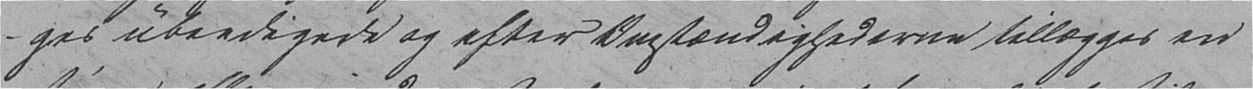ges ubeedigede og efter Omstændighederne tillægges en
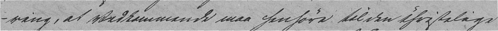ring, at Vedkommende maa henhøre til den christelige
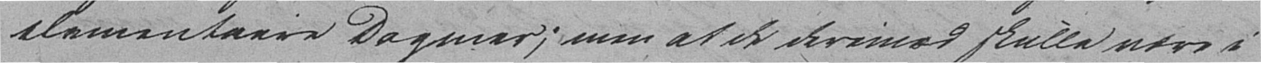elementaire Dogmer; men at de derimod skulle være i
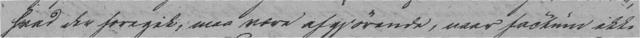hvad der foregik, maa være afgjørende, naar factum ikke
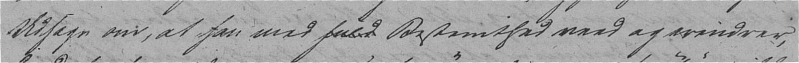Udsagn om, at han med fuld Bestemthed veed og erindrer,
